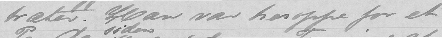træter. Han var heroppe for et
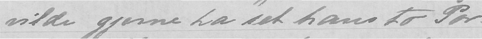vilde gjerne ha set hans to Por-
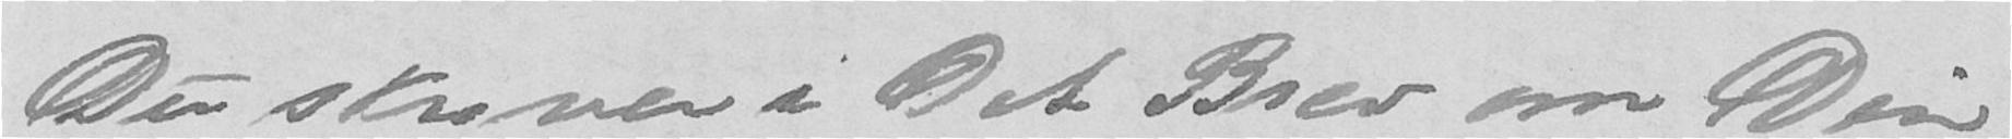Du skriver i Dit Brev om Din
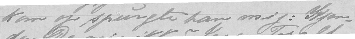kom op, spurgte han mig: Kjen-
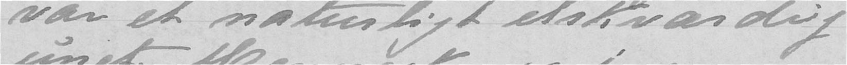var et naturligt elskværdig
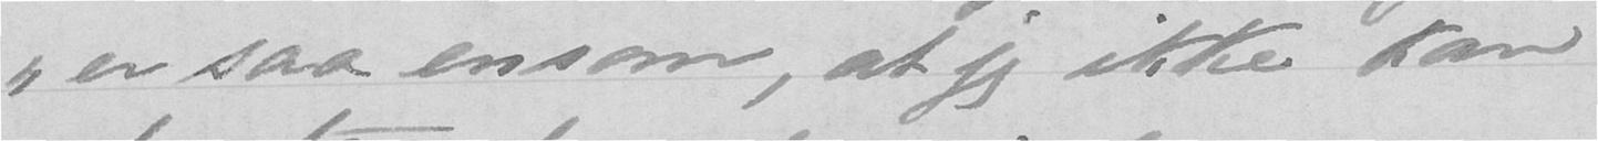" er saa ensom, at jeg ikke kan
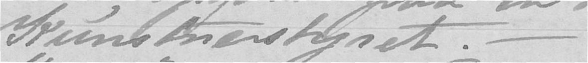Kunstnerstyret.
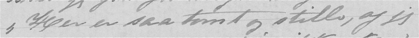" Her er saa tomt og stille, og jeg
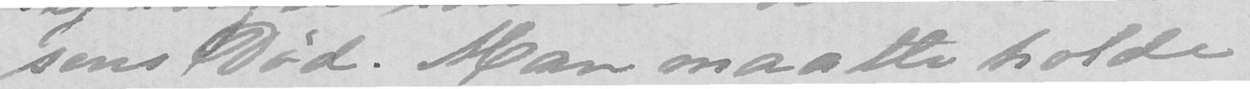sens Død. Man maatte holde
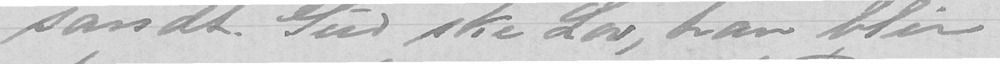sandt. Gud ske Lov, han blir
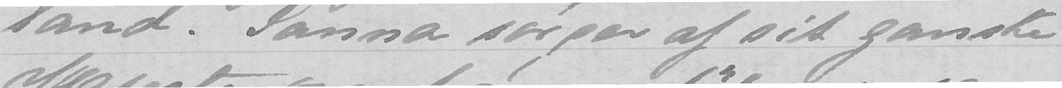land. Janna sørger af sit ganske
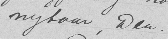nytaar, Dea.
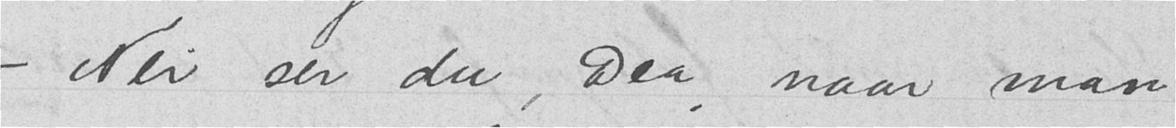- Nei ser du, Dea, naar man
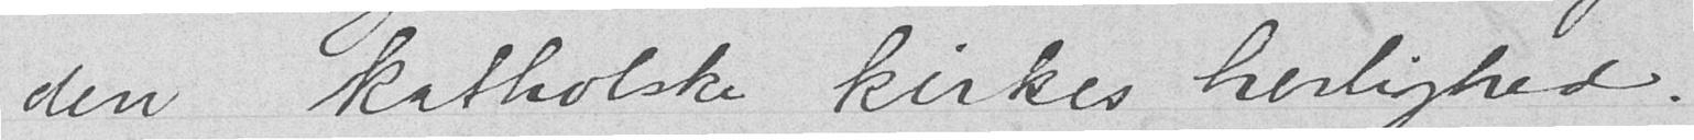den katholske kirkes herlighed.
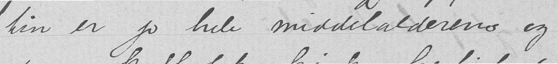tin er jo hele middelalderens og
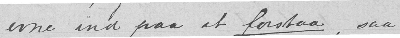evne ind paa at forstaa, saa
